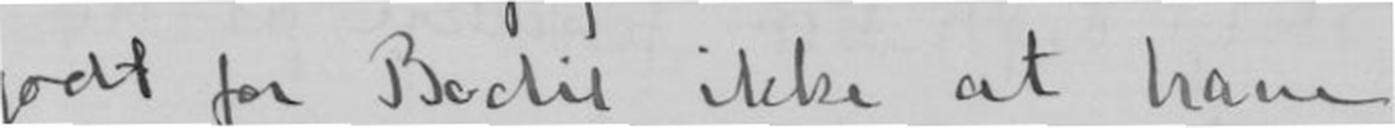godt for Bodil ikke at have
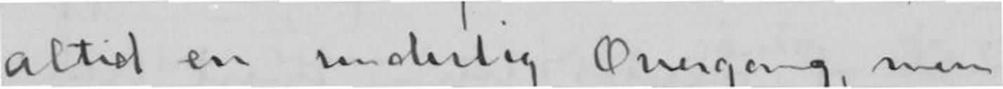altid en underlig Overgang, men
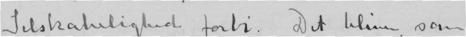Selskabelighed forbi. Det blive, som
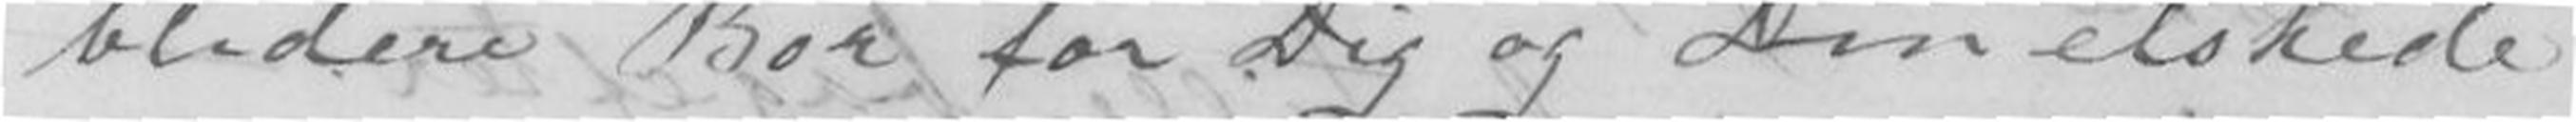blidere Bør for Dig og Din elskede
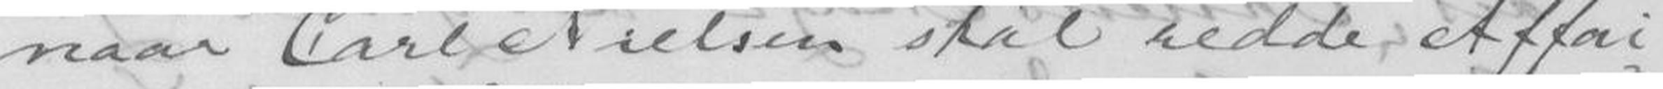naar Carl Nielsen skal redde Affai-
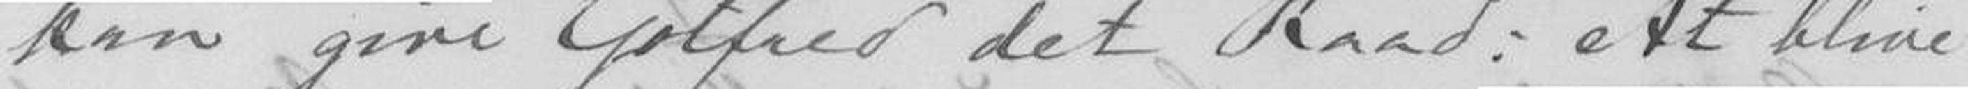kan give Gotfred det Raad: At blive
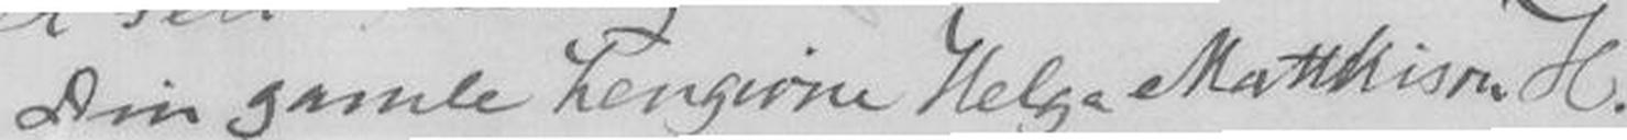Din gamle hengivne Helga Matthison H.
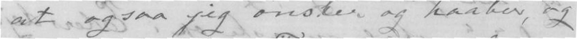at ogsaa jeg ønsker og haaber, og
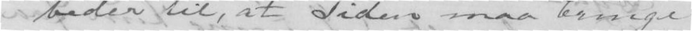beder til, at Tiden maa bringe
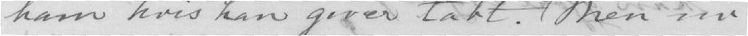ham hvis han giver tabt. Men nu
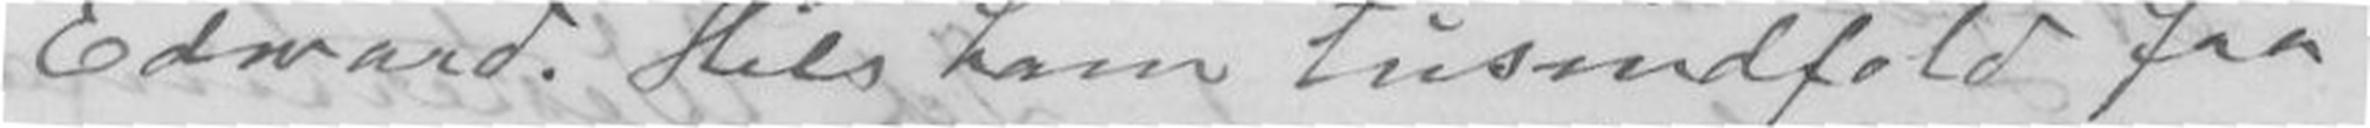Edward. Hils ham tusindfold fra
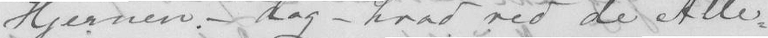Hjernen. - dog - hvad ved de Alle-
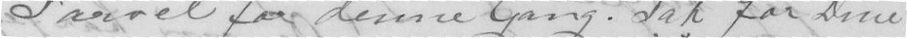Farvel for denne Gang. Tak for Dine
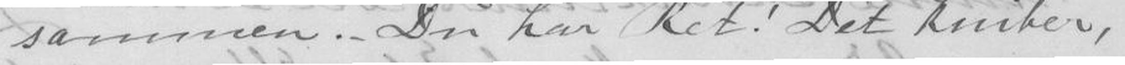sammen. - Du har Ret! Det kniber,
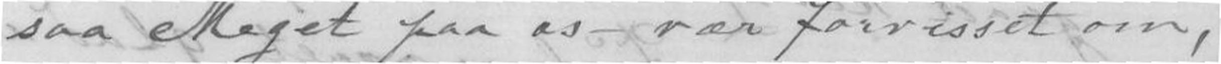saa Meget paa os - vær forvisset om,
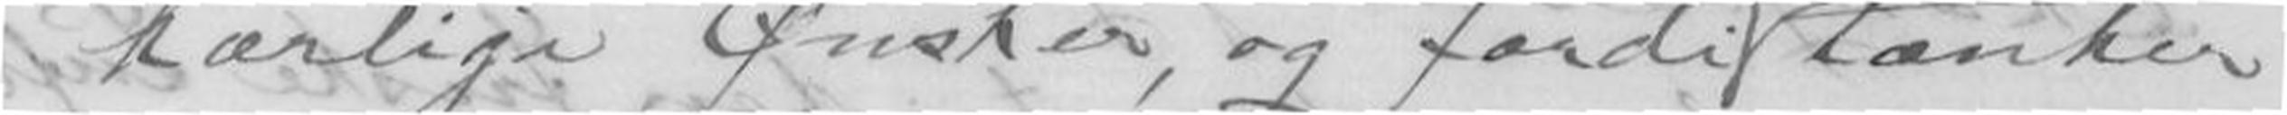kærlige Ønsker, og fordi tænker
